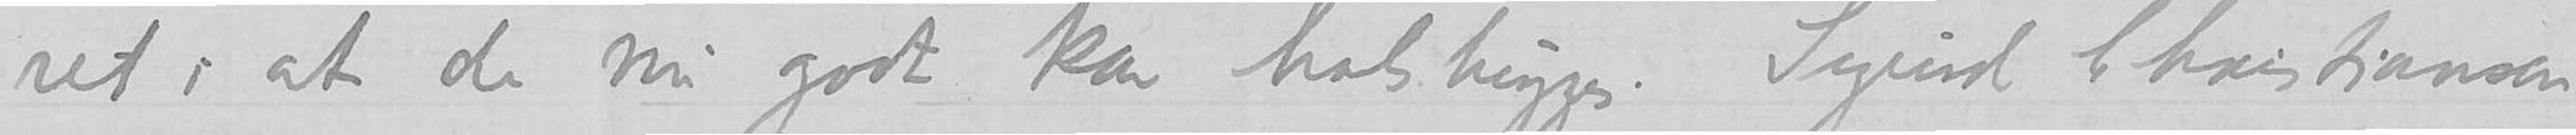ret i at de nu godt kan halshugges.  Sigurd Christiansen
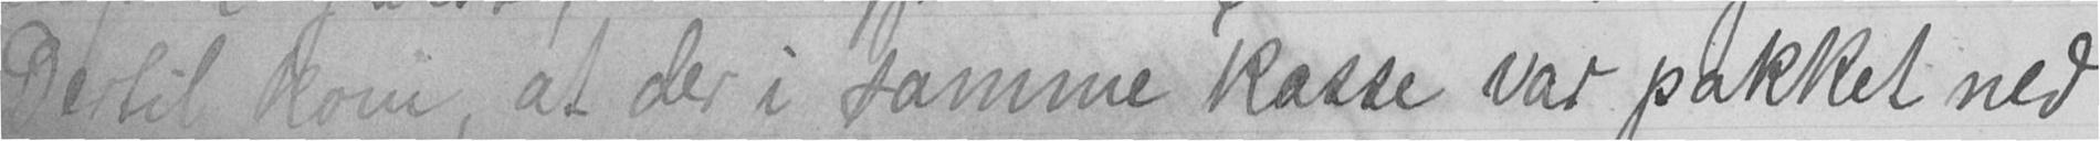Dertil kom, at der i samme kasse var pakket ned
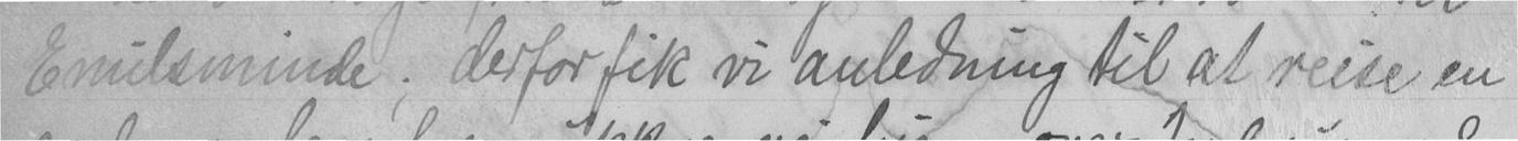Emilsminde; derfor fik vi anledning til at reise en
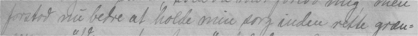forstod nu bedre at holde min sorg inden rette græn-
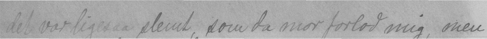det var ligesaa slemt, som da mor forlod mig, men
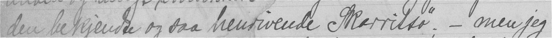den bekjendte og saa henrivende Skarridsø"; - men jeg
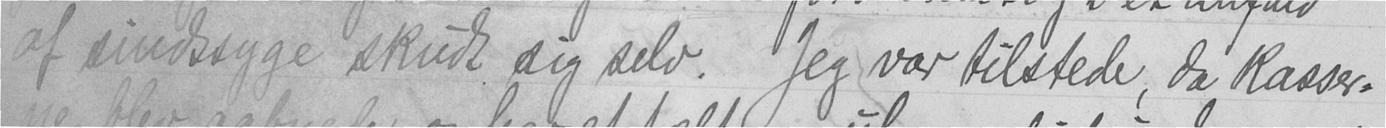af sindssyge skudt sig selv. Jeg var tilstede, da kasser-
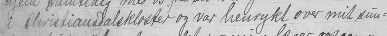i Christiansdalskloster og var henrykt over mit sun-
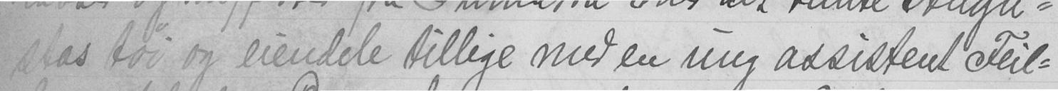stas tøi og eiendele tillige med en ung assistent Feil-
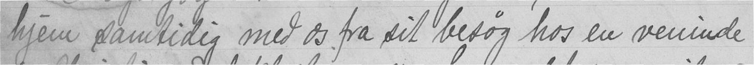hjem samtidig med os fra sit besøg hos en veninde
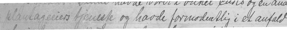plantageeiers tjeneste og havde formodentlig i et anfald
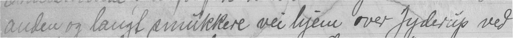anden og langt smukkere vei hjem over Jyderup ved
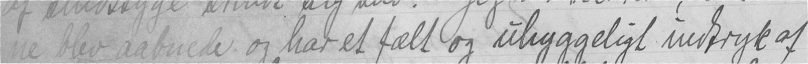ne blev aabnede og har et fælt og uhyggeligt indtryk af
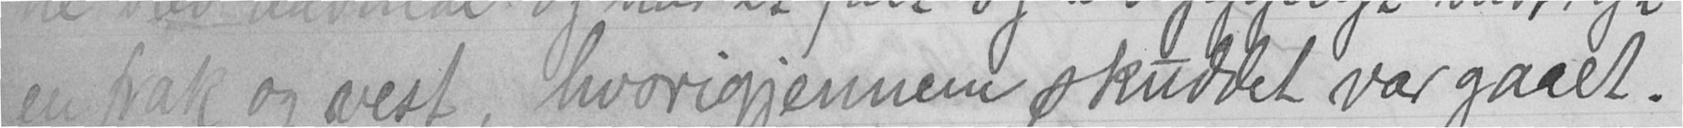en frak og vest, hvorigjennem skuddet var gaaet.
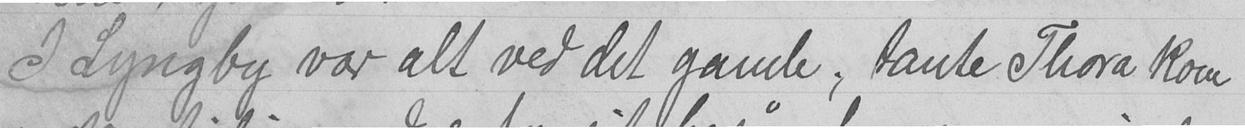I Lyngby var alt ved det gamle; tante Thora kom
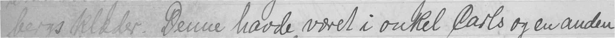bergs klæder. Denne havde været i onkel Carls og en anden
